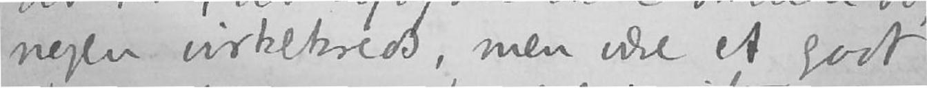nogen virkekreds, men være et godt
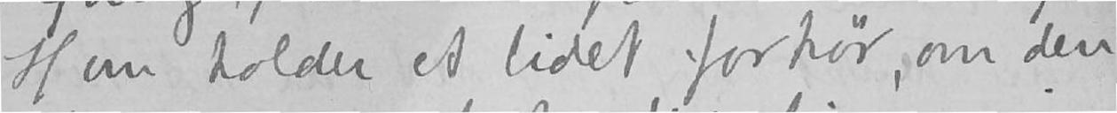Hun holder et lidet forhør, om den
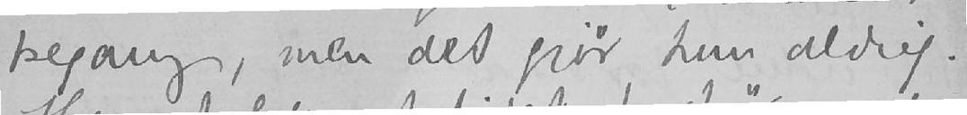kegang, men det gjør hun aldrig.
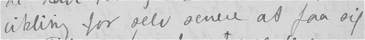vikling for selv senere at faa sig
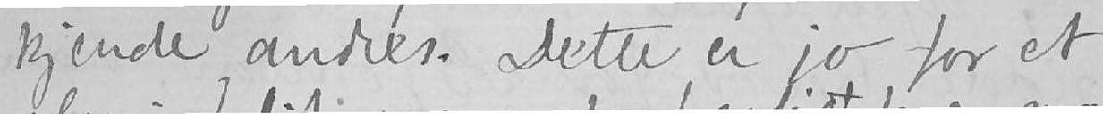kjende andres. Dette er jo for et
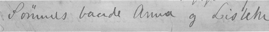Sømmes baade Anna og Lisbeth
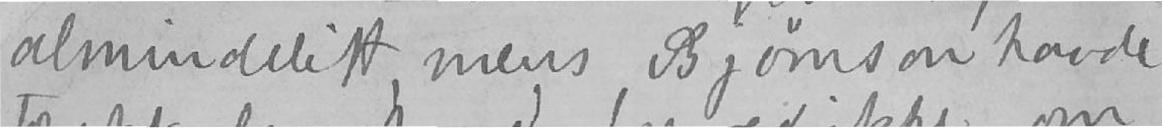almindeligt, mens Bjørnson havde
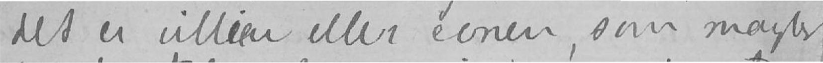det er villien eller evnen, som mangler
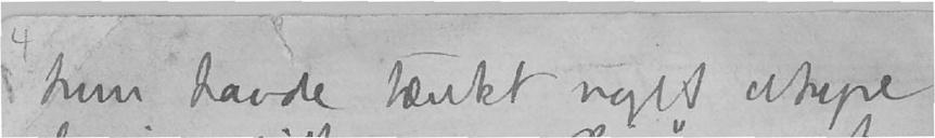hun havde tænkt noget uhyre
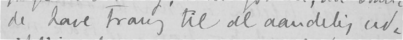have trang til aandelig ud-
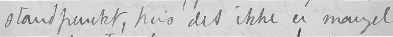standpunkt, hvis det ikke er mangel
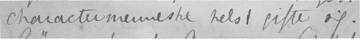charactermenneske helst gifte sig.
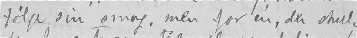følge sin smag, men for en, der skulde
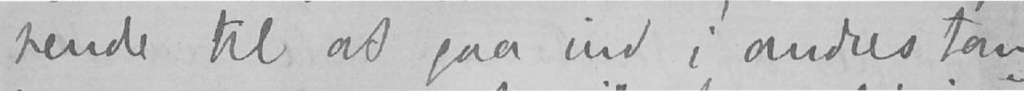hende til at gaa ind i andres tan-
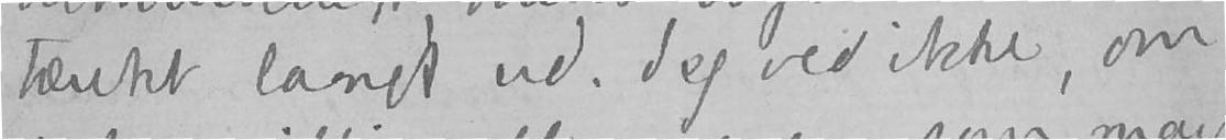tænkt langt ud. Jeg ved ikke, om
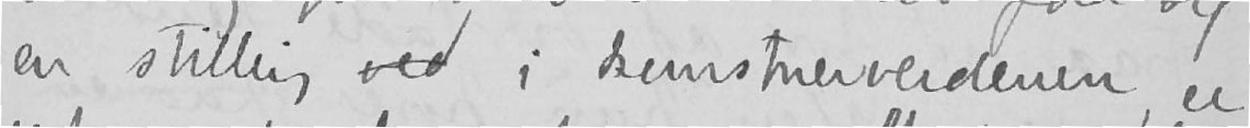en stilling ved i kunstnerverdenen, er
